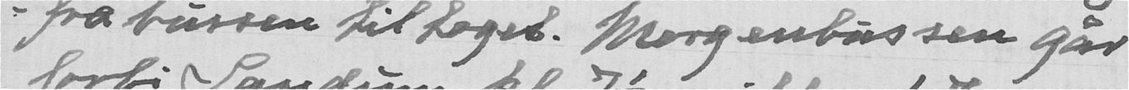- fra bussen til toget. Morgenbussen går
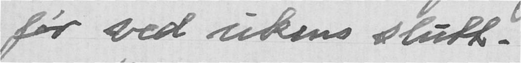før ved ukens slutt.
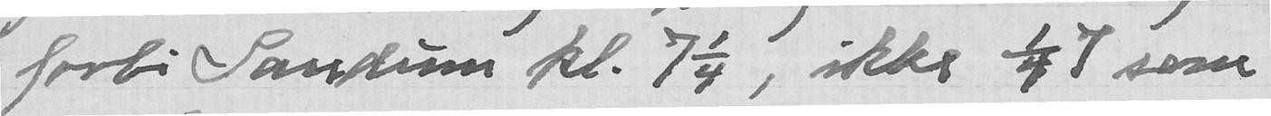forbi Sandum kl. 7 1/4, ikke 1/4 7 som
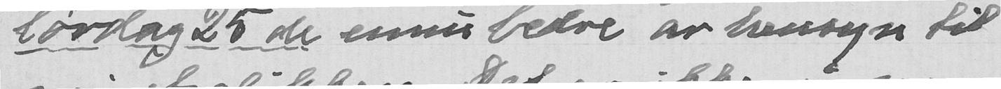lørdag 25de ennu bedre av hensyn til
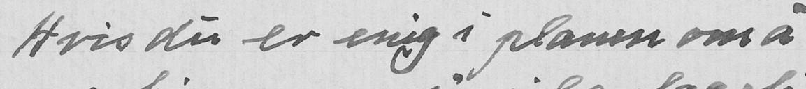Hvis du er enig i planen om å
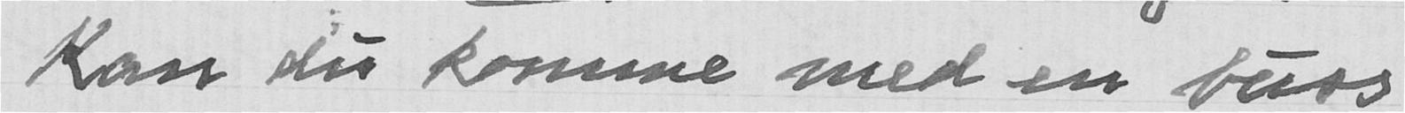Kan du komme med en buss
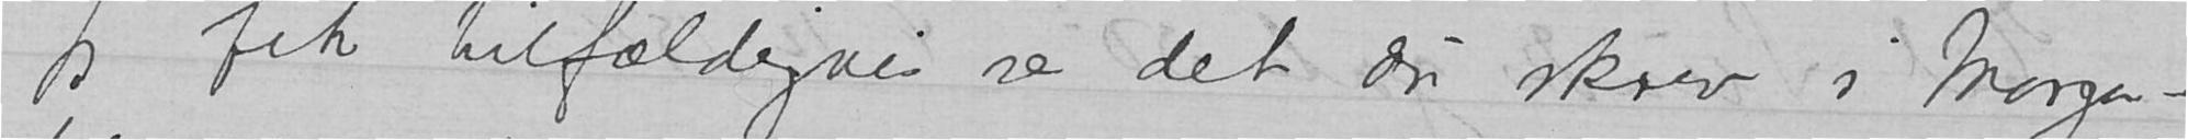Jeg fik tilfældigvis se det du skrev i Morgen
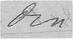Din
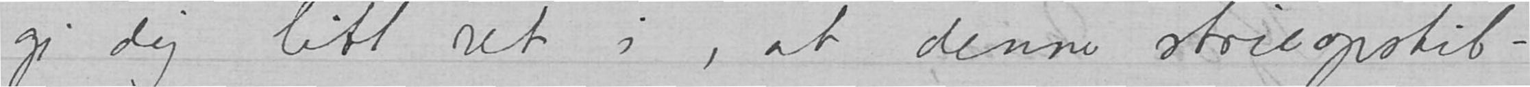gi dig litt ret i, at denne strie opstil
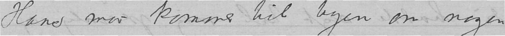Hans mor kommer til byen om nogen
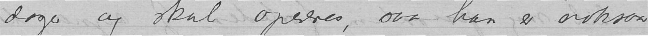dager og skal opereres, saa han er noksaa
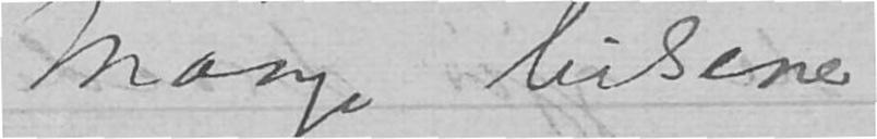Mange hilsener
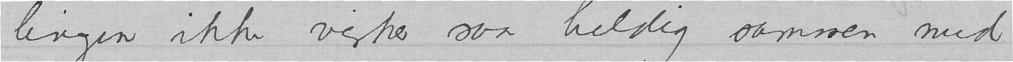lingen ikke virker saa heldig sammen med
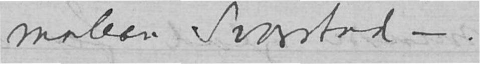maleren Svarstad –.
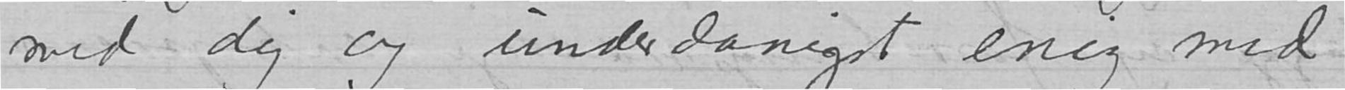med dig og underdanigst enig med
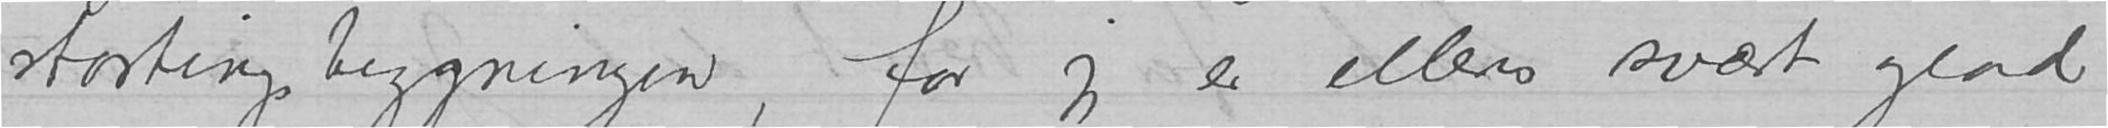stortingsbygningen, for jeg er ellers svært glad
